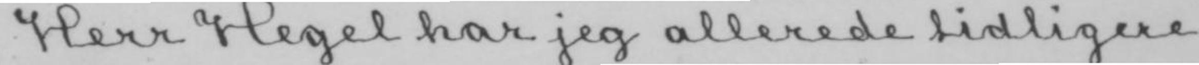Herr Hegel har jeg allerede tidligere
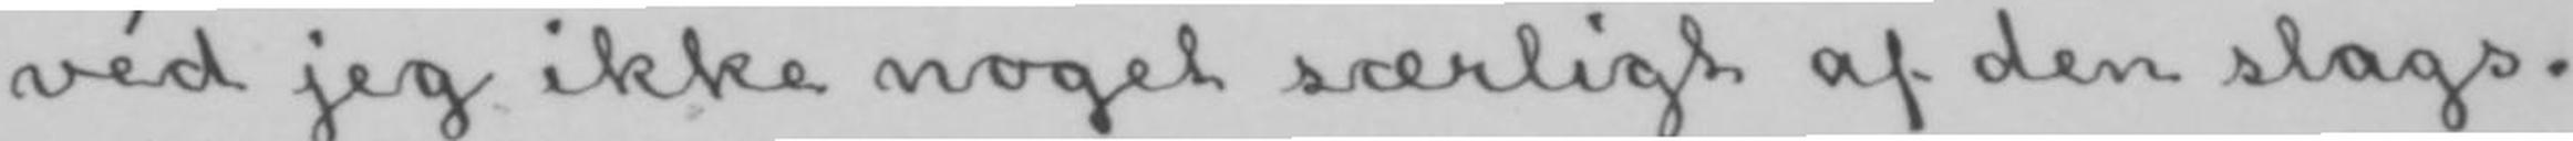véd jeg ikke noget særligt af den slags.
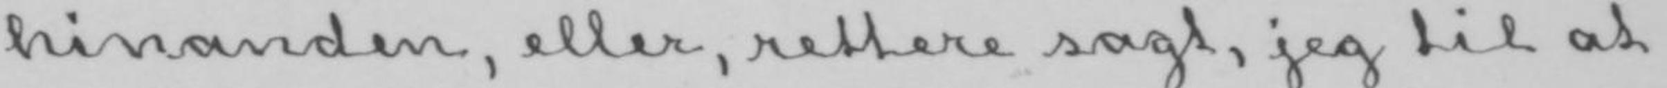hinanden, eller, rettere sagt, jeg til at
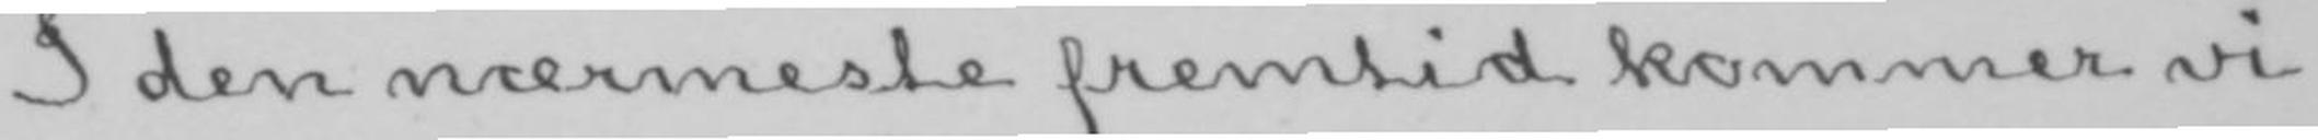I den nærmeste fremtid kommer vi
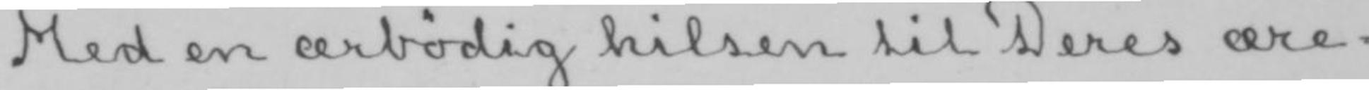Med en ærbødig hilsen til Deres ære-
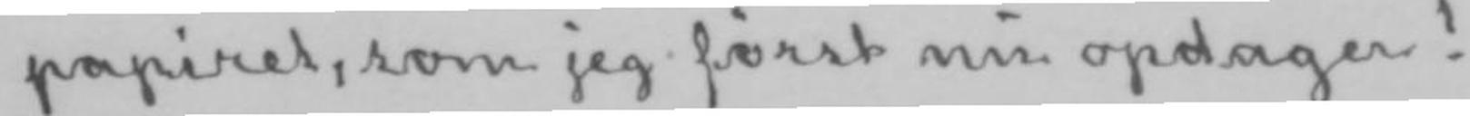papiret, som jeg først nu opdager!
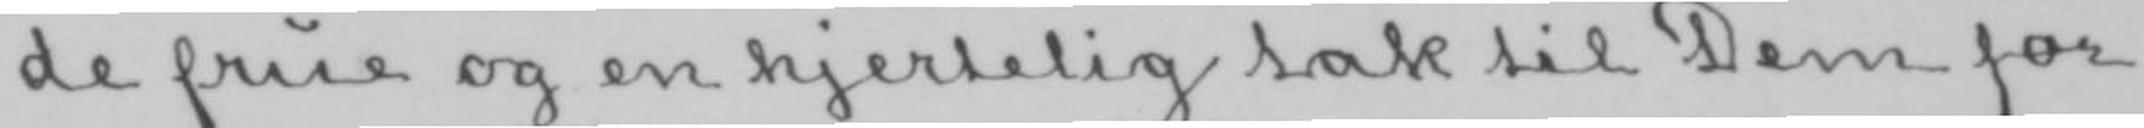de frue og en hjertelig tak til Dem for
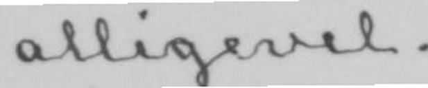alligevel.
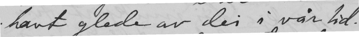havt glede av dei i vår tid.
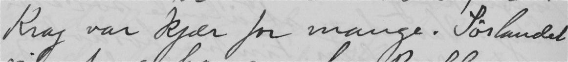Krag var kjær for mange. Sörlandet
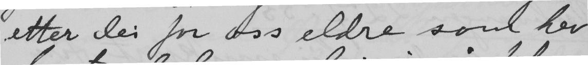etter dei for oss eldre som hev
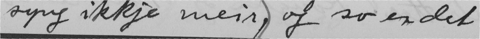syng ikkje meir, og so er det
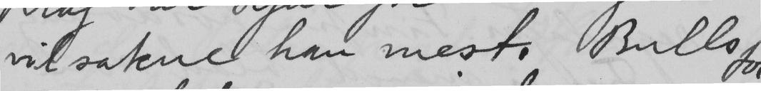vil sakne han mest. Bulls
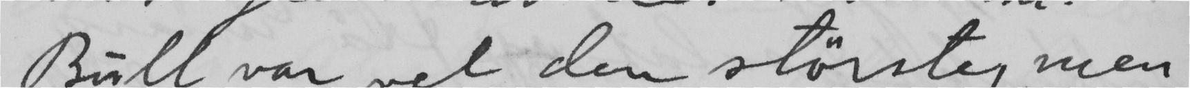Bull var vel den störste, men
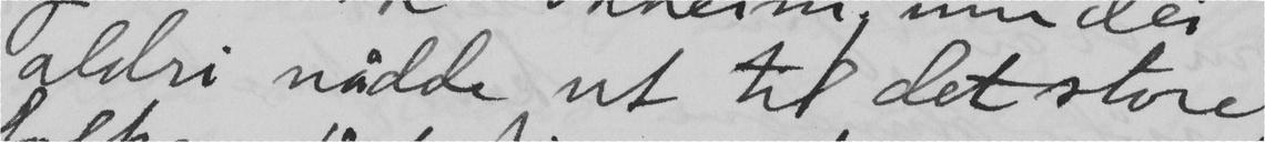aldri nådde ut til det store
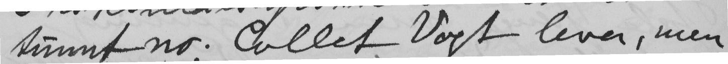tunnt no. Collet Vogt lever, men
